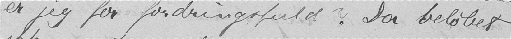er jeg for fordringsfuld? Da beløbet
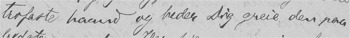trofaste haand og beder Dig greie den paa
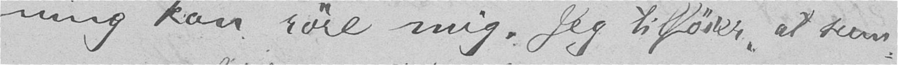ning kan røre mig. Jeg tilføier, at sum-
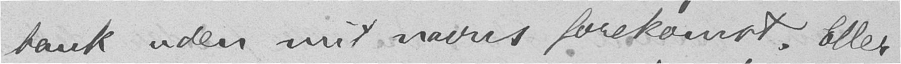bank uden mit navns forekomst. Eller
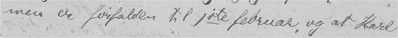men er forfalden til 1ste februar, og at Karl
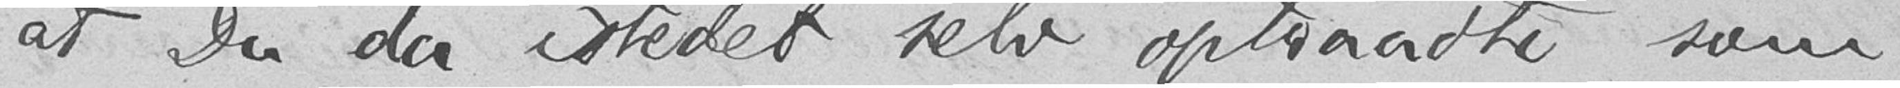at Du da istedet selv optraadte som
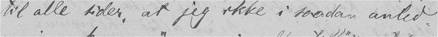til alle sider, at jeg ikke i saadan anled-
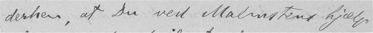derhen, at Du ved Malmstens hjælp
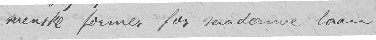svenske former for saadanne laan
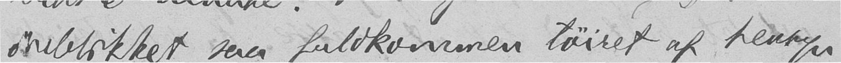øieblikket saa fuldkommen tøiset af hensyn
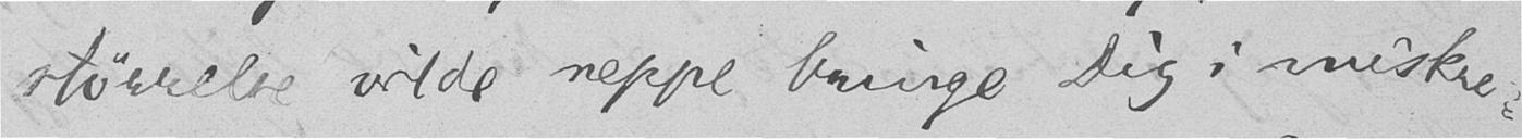størrelse vilde neppe bringe Dig i miskre-
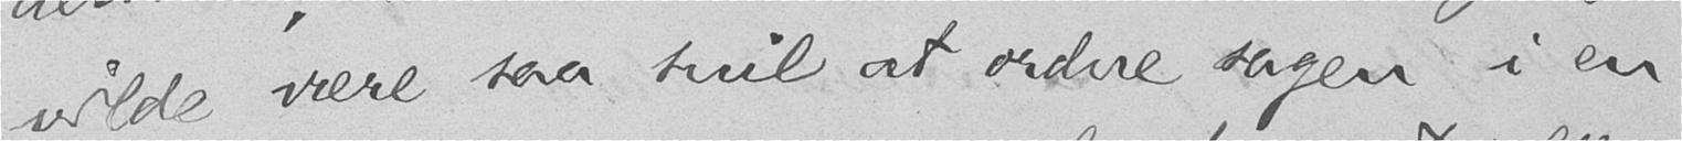vilde være saa snil at ordne sagen i en
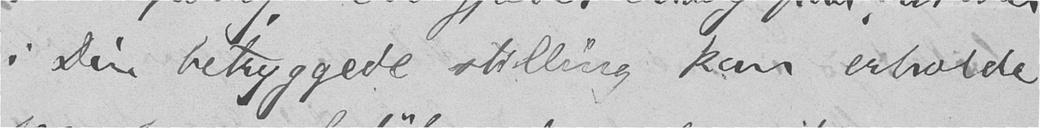i Din betryggede stilling kan erholde
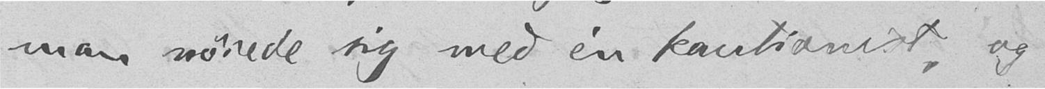man nøiede sig med en kautionist, og
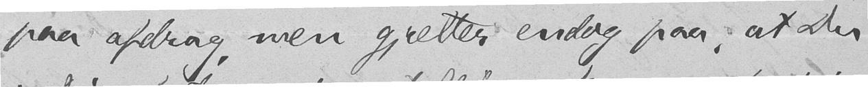paa afdrag, men gjætter endog paa, at Du
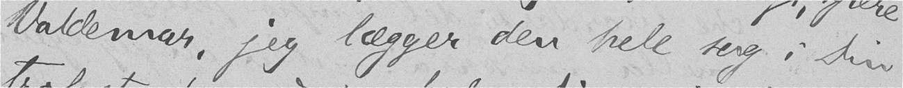Valdemar, jeg lægger den hele sag i Din
